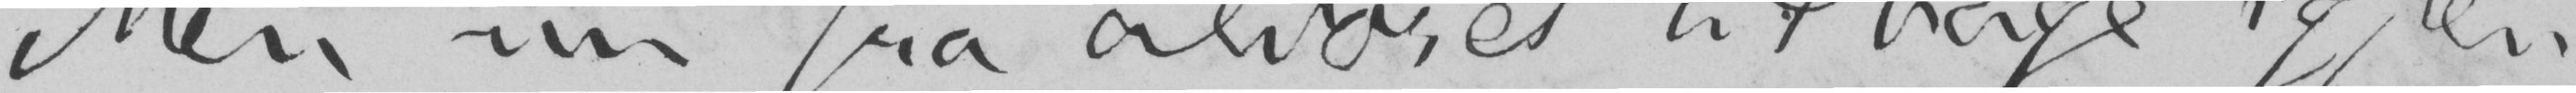Men nu fra alvoret tilbage igjen
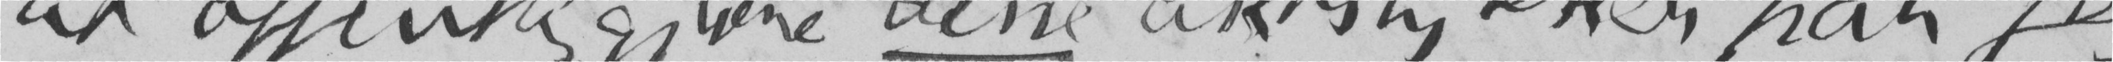at offentliggjøre dine aktstykker har jeg
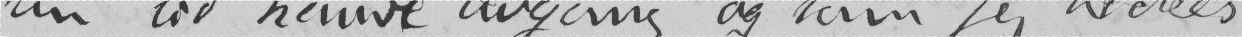sin tid havde adgang, og som jeg tildels
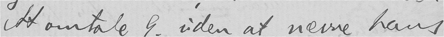At omtale G. uden at nævne hans
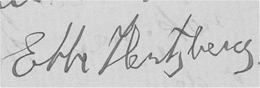Ebbe Hertzberg.
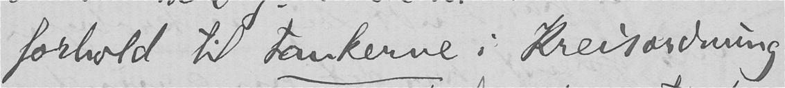forhold til tankerne i Kreisordnung
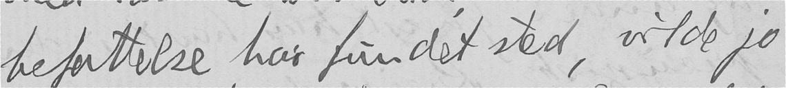befattelse har fundet sted, vilde jo
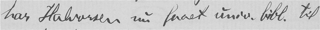har Halvorsen nu faaet univ.bibl. til
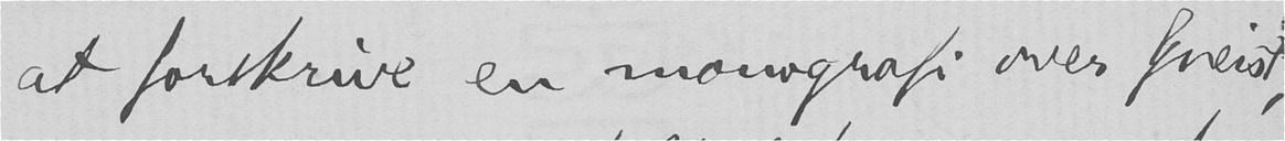at forskrive en monografi over Gneist,
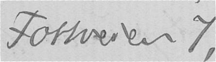Fossveien 7,
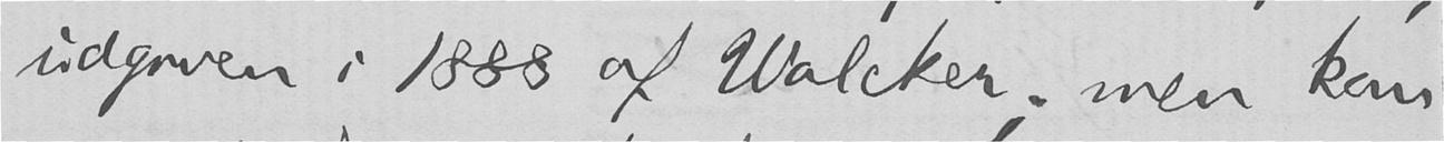udgiven i 1888 af Walcker; men kan
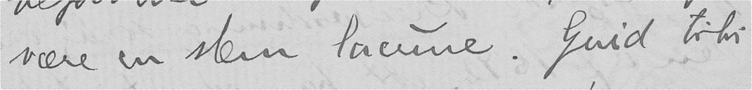være en slem lacune. Quid tibi
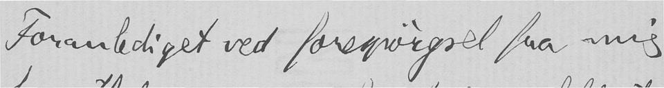Foranlediget ved forespørgsel fra mig,
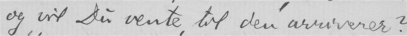og vil Du vente, til den arriverer?
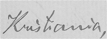Kristiania,
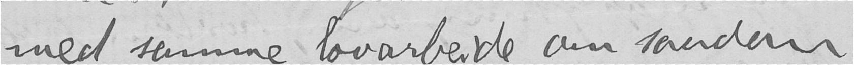med samme lovarbeide, om saadan
